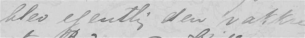blev egentlig den vakkre-
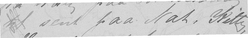til sent paa Nat. Kitty
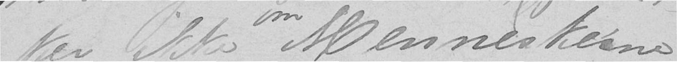ker ikke om Menneskerne
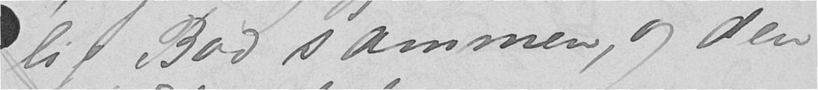lig Bod sammen, og den
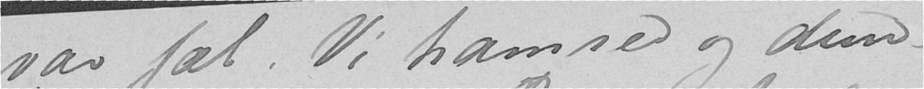var fæl. Vi hamred og dun-
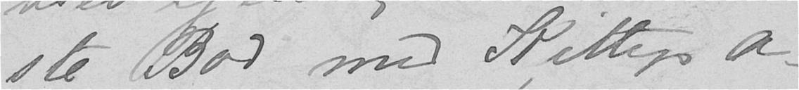ste Bod med Kittys a-
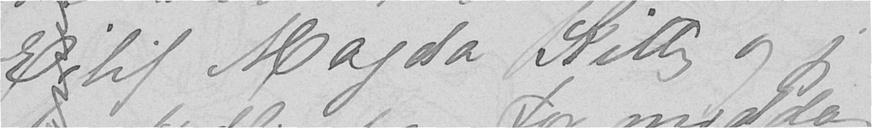Eilif Magda Kitty og jeg
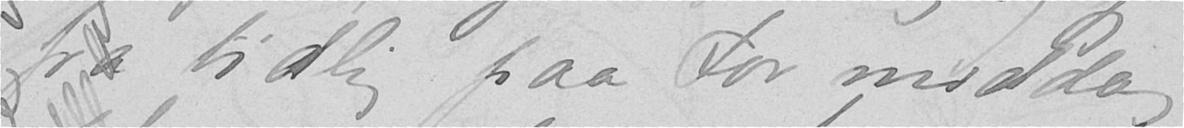fra tidlig paa Formiddag
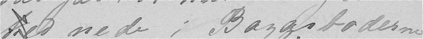ked nede i Bazarboderne
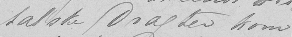talske Dragter kom
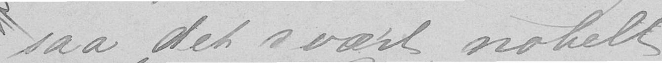saa det svært nobelt
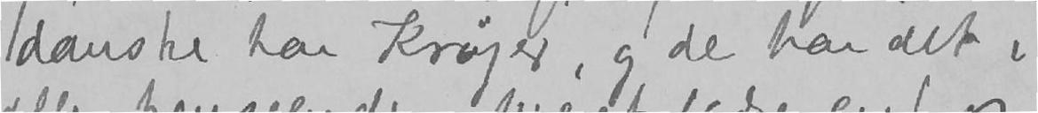danske har Krøyer, og de har det i
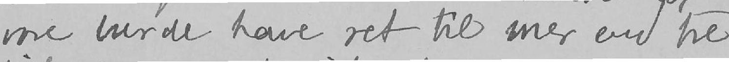vore burde have ret til mer end tre
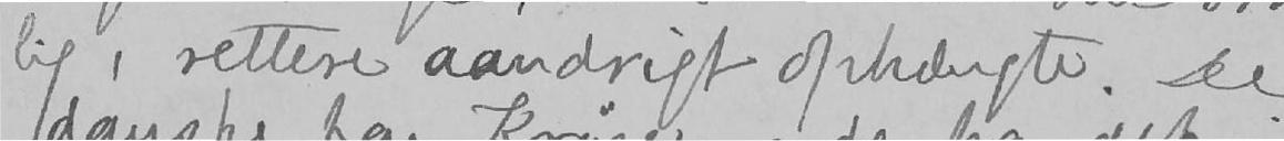lig, rettere aandrigt ophængte. De
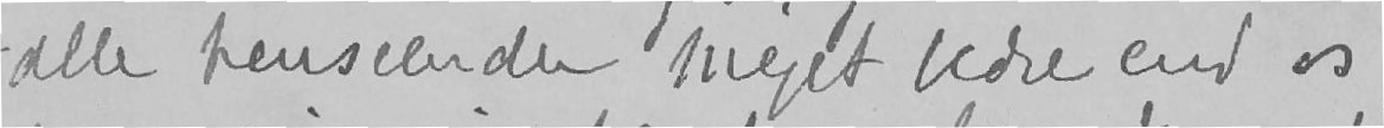alle henseender meget bedre end os,
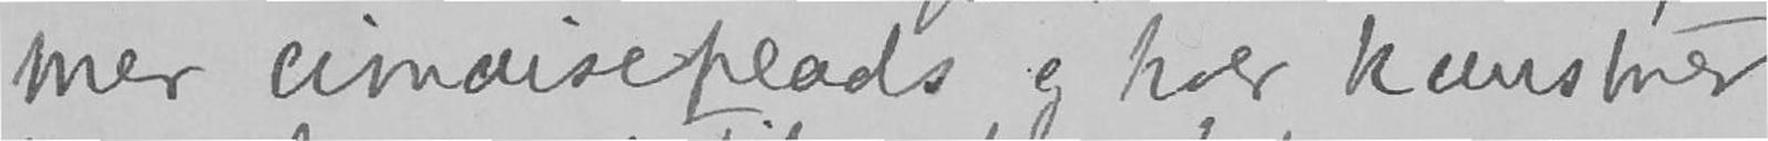mer cimaiseplads og hver kunstner
